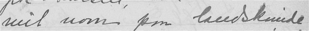mit navn paa landskvinde
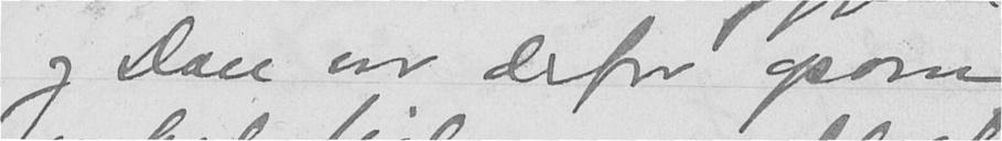og Don var derfor opom
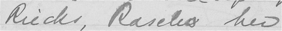Riecks, Raschs her
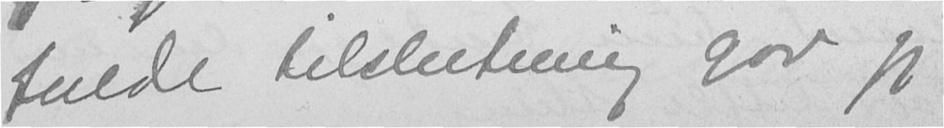fulde tilslutning gav jeg
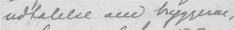udtalelse om bryggerne,
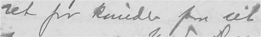ret for kvinder paa sit
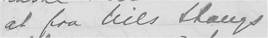at faa Nils Stangs
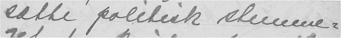sætte politisk stemme-
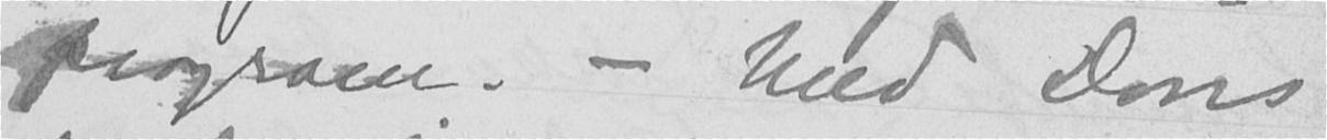program. - Med Dons
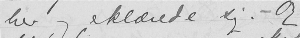her og eklærede sig. - Og
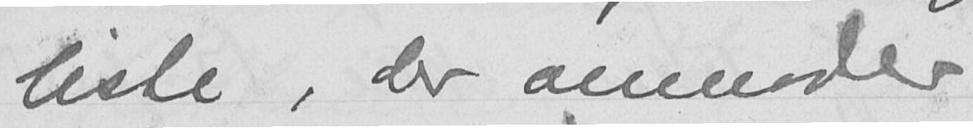liste, der anmoder
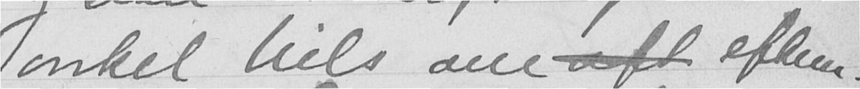onkel Nils om aft efterm.
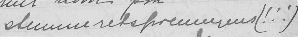stemmeretsforeningens (!!!)
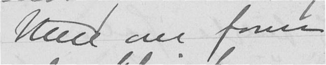Men om form
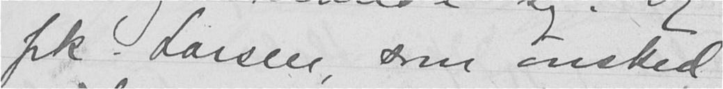frk. Larsen, som ønsked
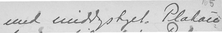med middagstoget. Platou
